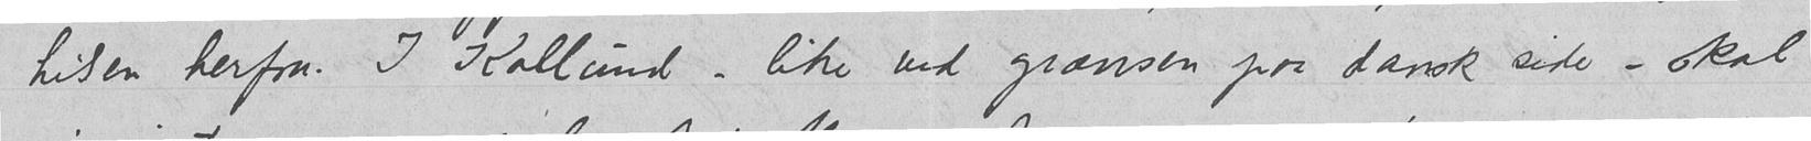hilsen herfra. I Kallund – like ved grænsen paa dansk side – skal
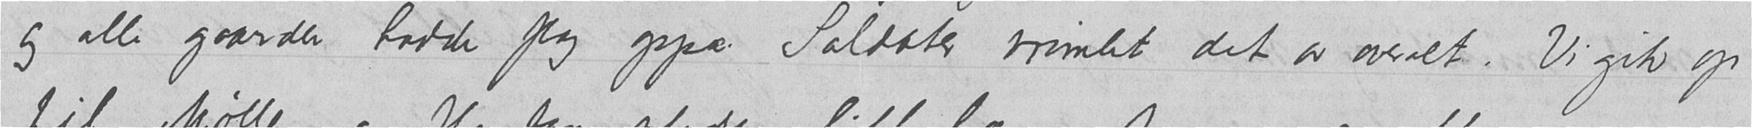og alle gaarder hadde flag oppe. Soldater vrimlet det av overalt. Vi gik op
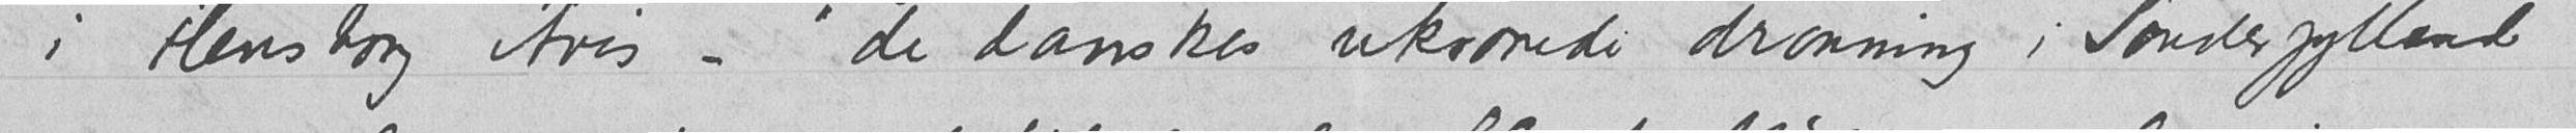i Flensborg Avis – "de danskes ukronede dronning i Sønderjylland"
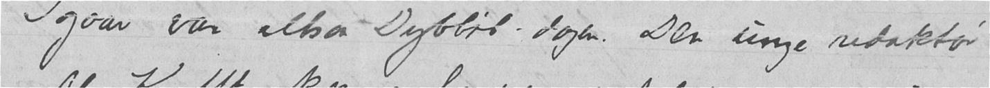Igaar var altsaa Dybbøl-dagen. Den unge redaktør
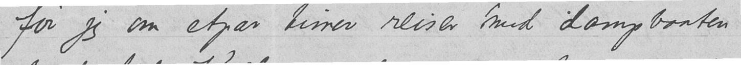før jeg om etpar timer reiser med dampbaaten
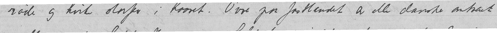røde og hvite sløifer i haaret. Bare paa fastlandet er alle danske omtrent
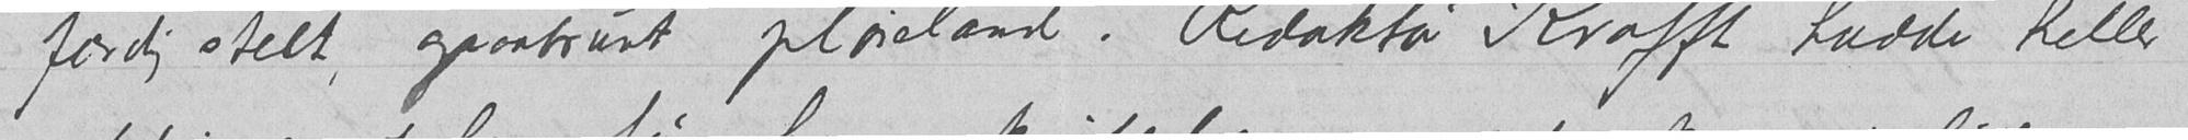færdig stelt, graabrunt pløieland. Redaktør Krafft hadde heller
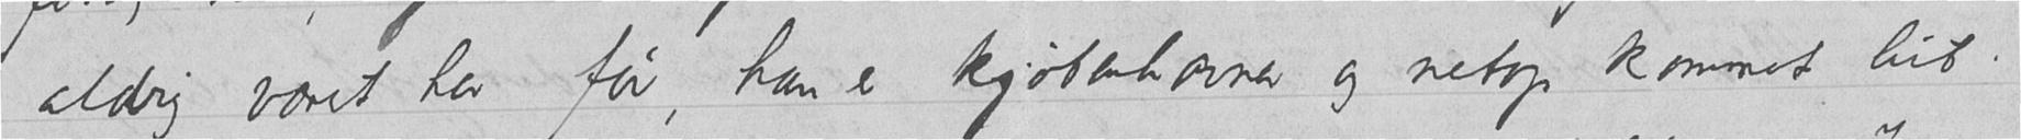aldrig været her før, han er kjøbenhavner og netop kommet hit.
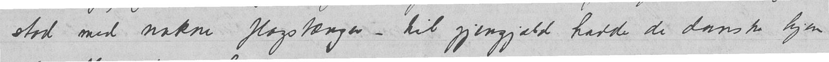stod med nakne flagstænger – til gjengjæld hadde de danske hjem
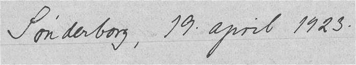Sønderborg, 19. april 1923.
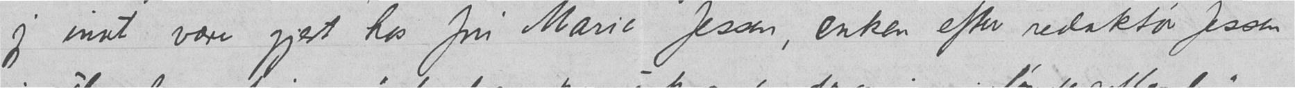jeg inat være gjest hos fru Marie Jessen, enken efter redaktør Jessen
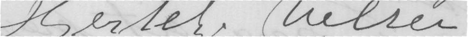Hjertelig hilsen
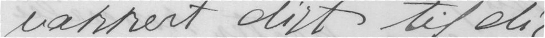vakkert digt til dig
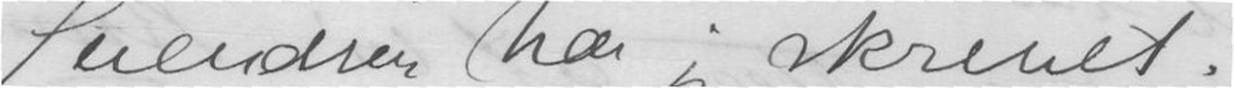Svendsen har jeg skrevet.
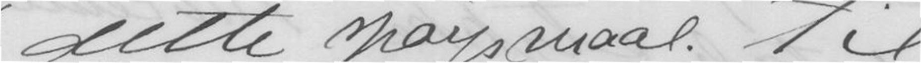dette spørgsmaal til
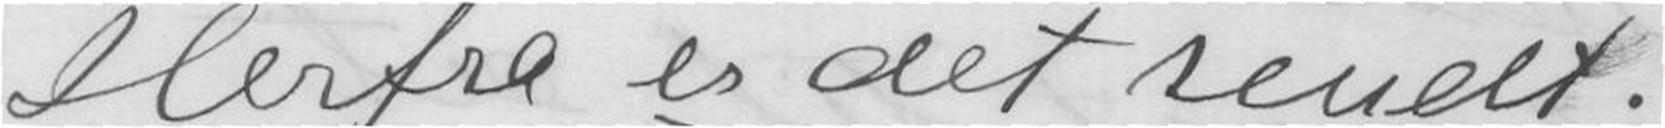Herfra er det sendt.
